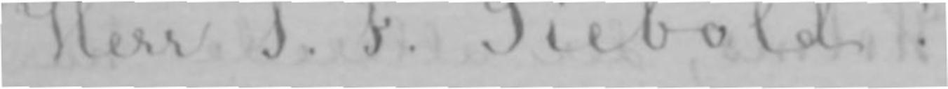Herr P. F. Siebold!
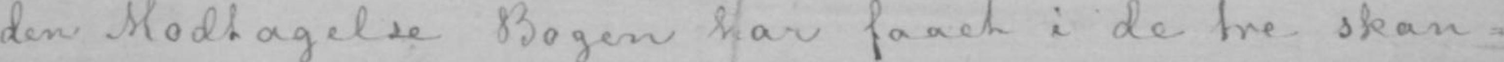den Modtagelse Bogen har faaet i de tre skan-
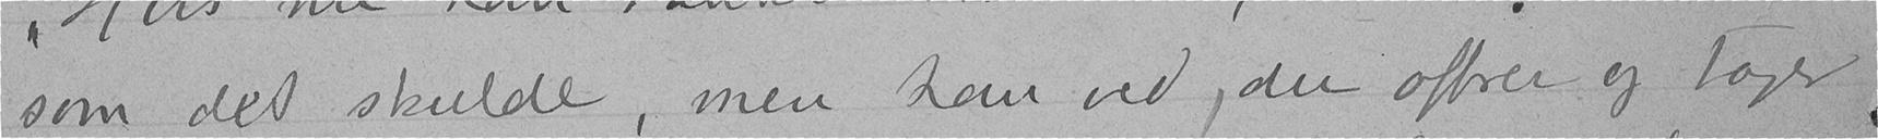som det skulde, men han ved, du offrer og tager
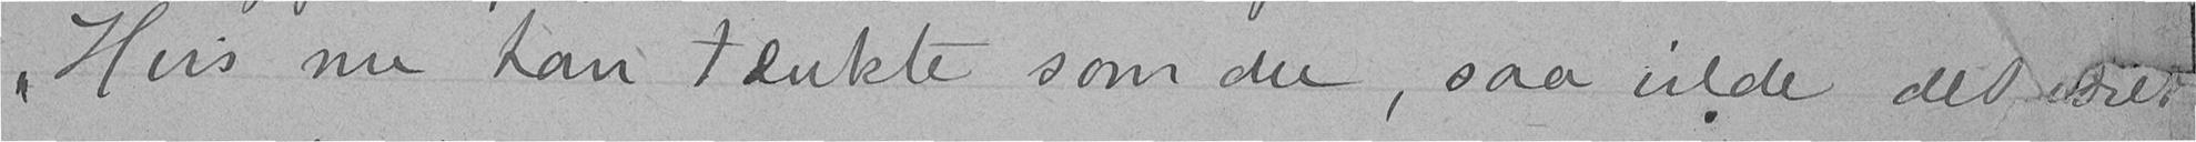"Hvis nu han tænkte som du, saa vilde det været
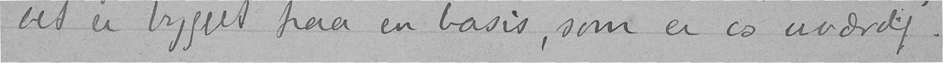bet er bygget paa en basis, som er os uværdig.
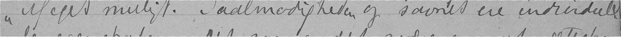"Meget muligt. Taalmodigheden og savnet ere individuel-
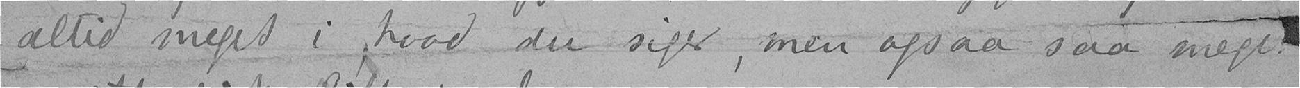altid meget i hvad du siger, men ogsaa saa meget
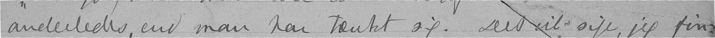anderledes, end man har tænkt sig. Det vil sige, jeg fin-
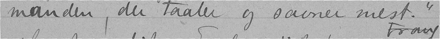manden, der taaler og savner mest."
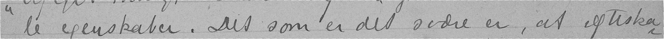le egenskaber. Det som er det svære er, at ægteska-
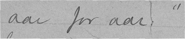aar for aar."
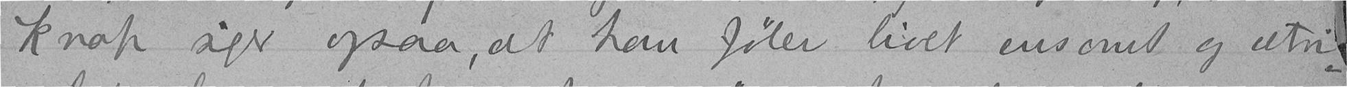Knop siger ogsaa, at han føler livet ensomt og utri-
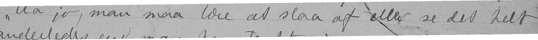"Aa jo, man maa lære at slaa af eller se det helt
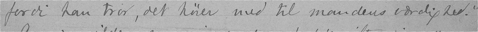fordi han tror, det hører med til mandens værdighed."
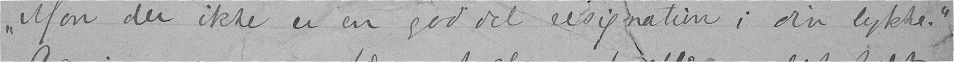"Mon der ikke er en god del resignation i din lykke."
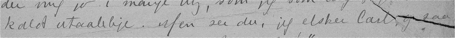kaldt utaalelige. Men ser du, jeg elsker Carl, og saa
